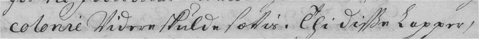colonie Videre skulde sættis. Thi disse Lapper,
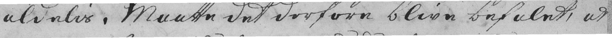aldelis. Maatte det derfore blive befalet, at
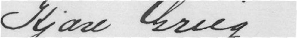Kjære Grieg!
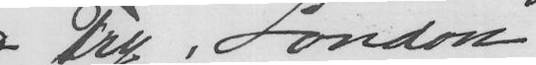& Fry, London
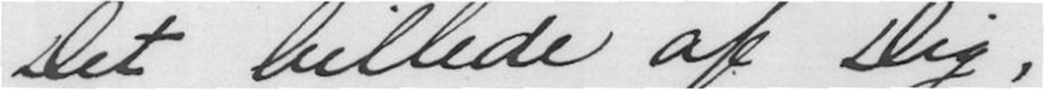Det billede af Dig,
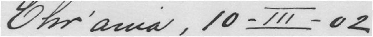Chr'ania, 10-III-02.
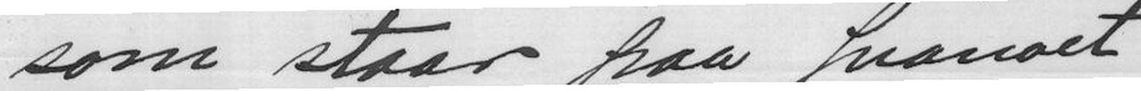som staar paa pianoet
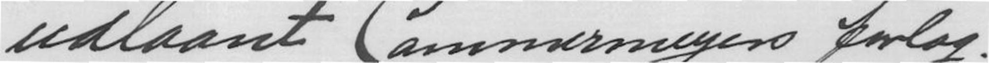udlaant Cammermeyer forlag.
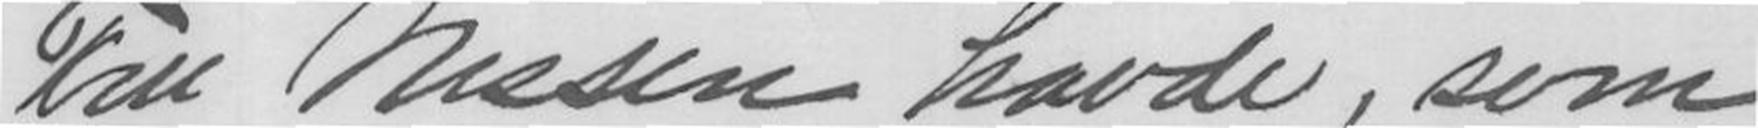Fru Nissen havde, som
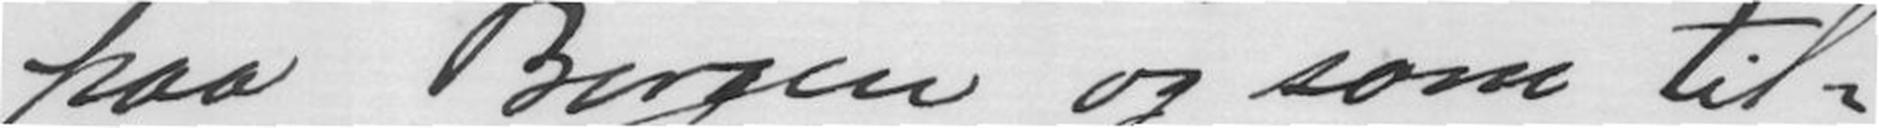paa Borgen og som til-
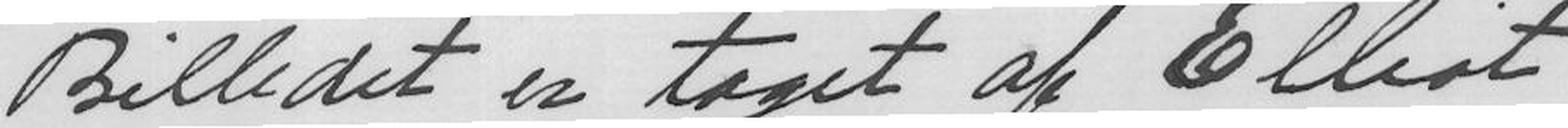Billedet er taget af Elliot
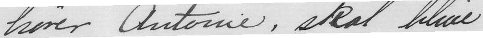hører Antonie, skal blive
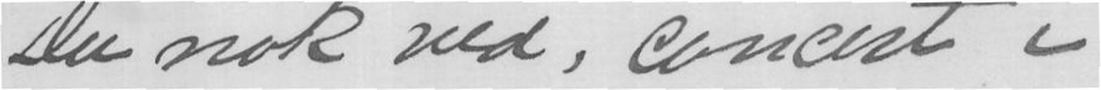Du nok ved, concert i
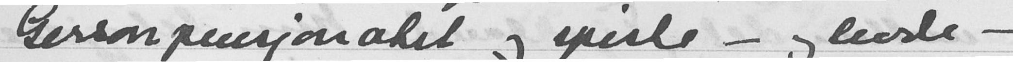Gersonpensjonatet og spiste - og levde -
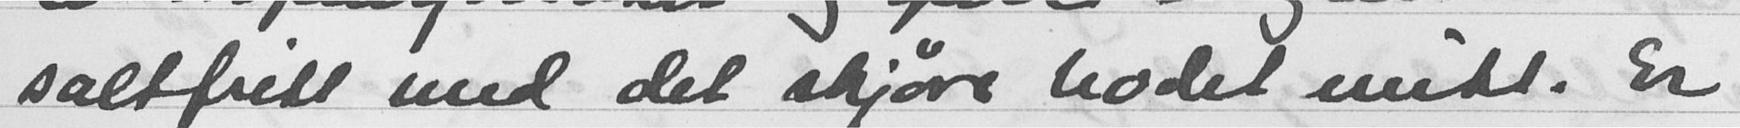saltfritt med det skjøre hodet mitt. Er
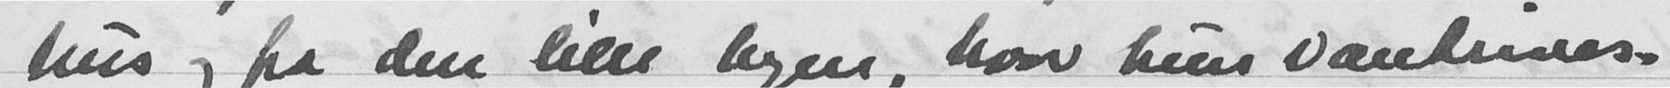hus og fra den lille byen, hvor huns vantrives.
